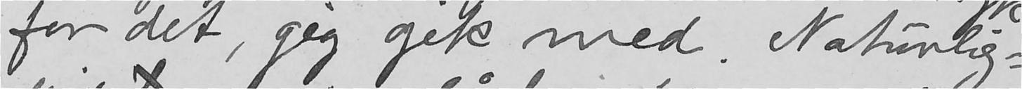for det, jeg gik med. Naturlig-
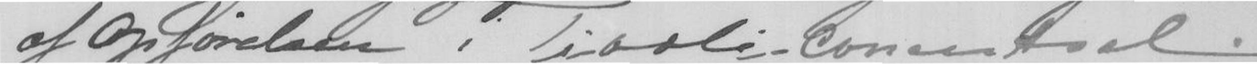af Opførelsen i Tivoli-Concertsal.
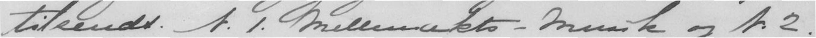tilsendt N.1.Mellemcekts-Musik og N.2.
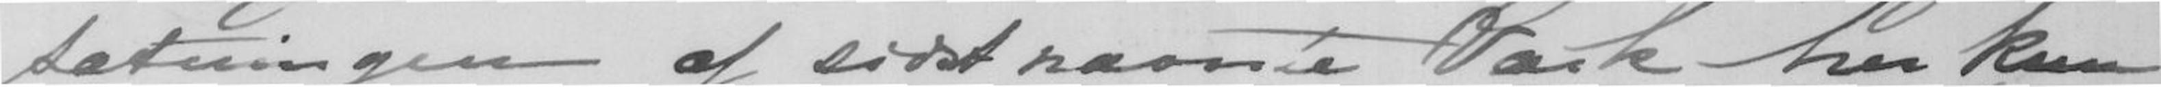sætningen af sidst nævnte Værk her kun
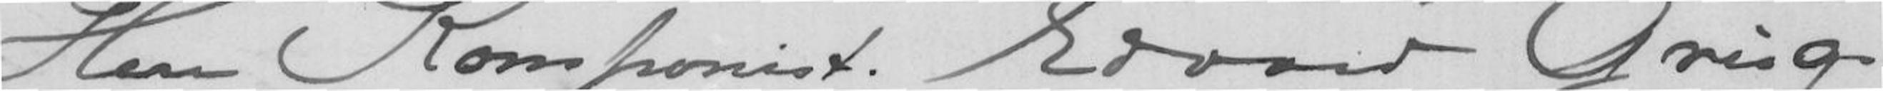Herr Komponist. Edvard Grieg.
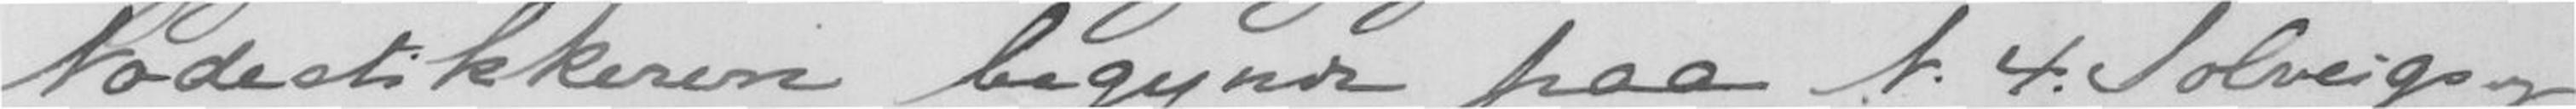Nodestikkeren begynde paa N.4. Solveigs og
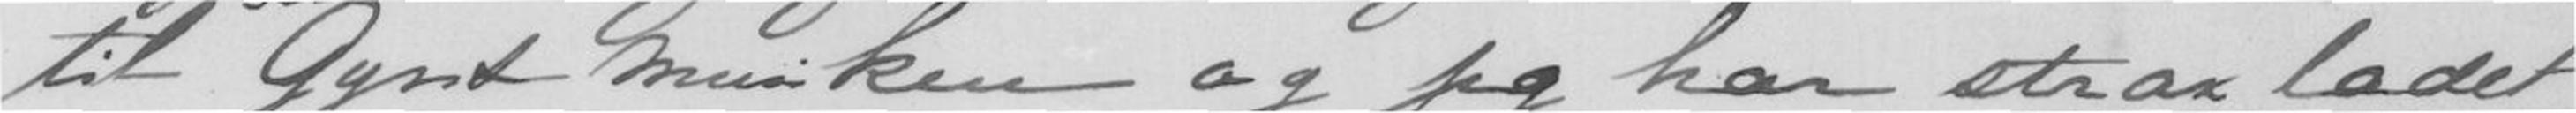til Per Gynt Musiken og jeg har strax ladet
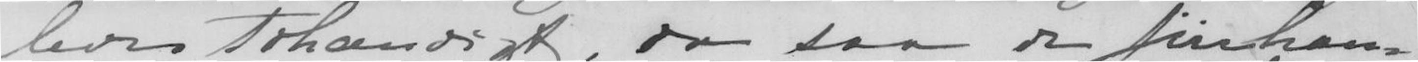ledes Tohændigt, da saa de fiirhændige
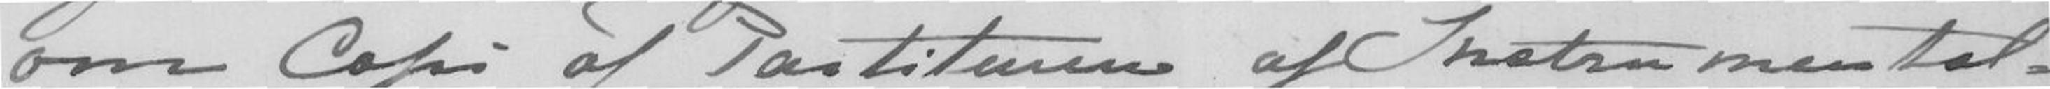om Copi af Partituren af Instrumental-
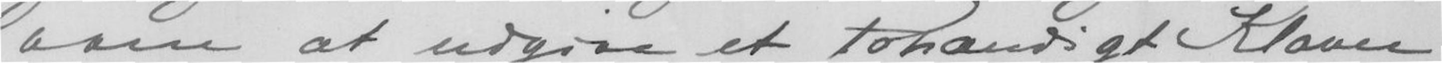vane at udgive et tohændigt Klaver-
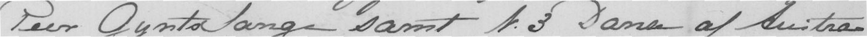Per Gynts Sange samt N.3 Dance af Anitra
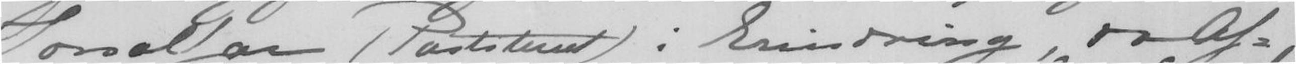Jorsalfar (Partituret) i Erindring, da Af-
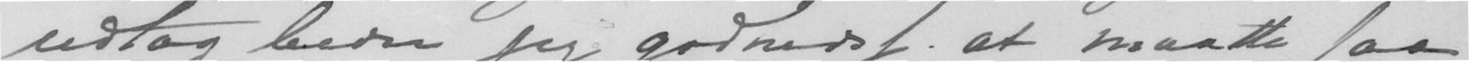udtag beder jeg godhedsf. at maatte faa
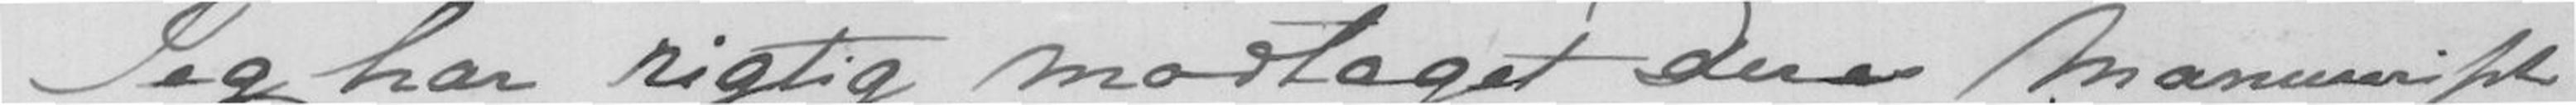Jeg har rigtig mogtaget Deres Manuscript
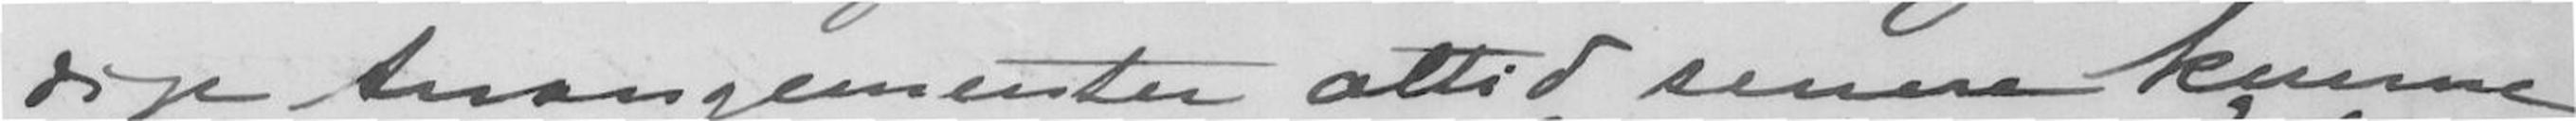Arrangementer altid senere kunne
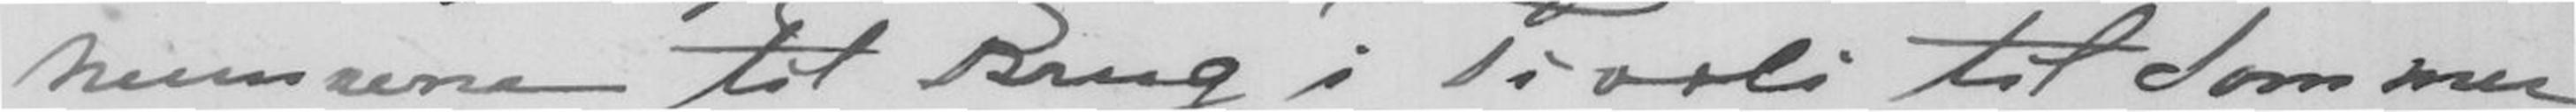numrene til Brug i Tivoli til Sommer
